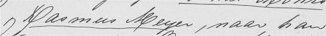og Rasmus Meyer, naar han
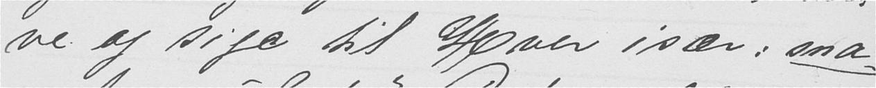ve og sige til Hver især, sna-
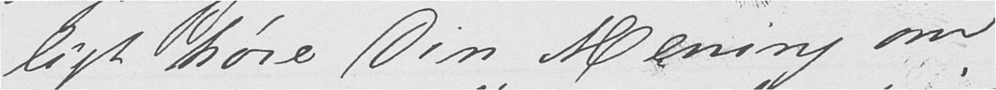ligt høre Din Mening om
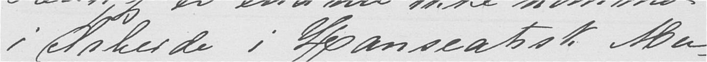i Arbeide i Hanseatisk Mu-
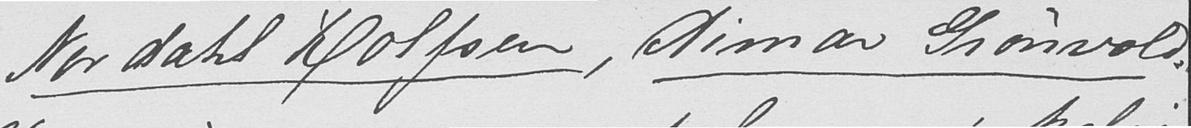Nordahl Rolfsen, Aimar Grønvold.
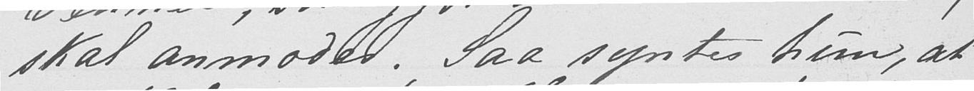skal anmodes. Saa syntes hun, at
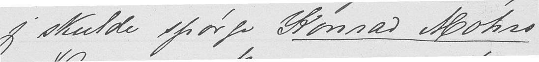jeg skulde spørge Konrad Mohrs
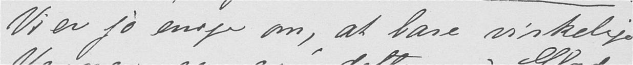Vi er jo enige om, at bare virkelige
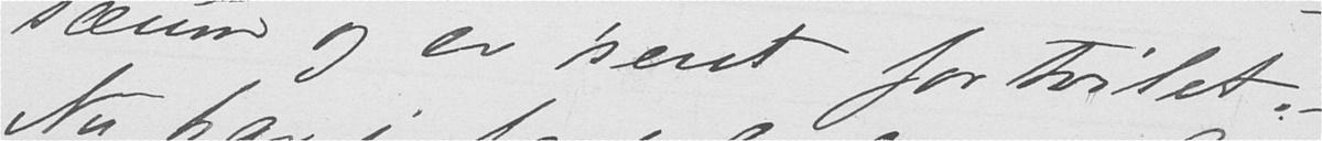sæum og er rent fortvilet.
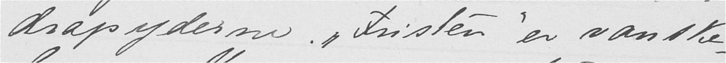dragsyderne. "Fristen" er vanske-
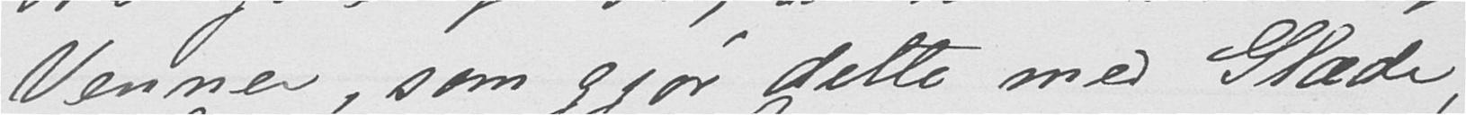Venner, som gjør dette med Glæde,
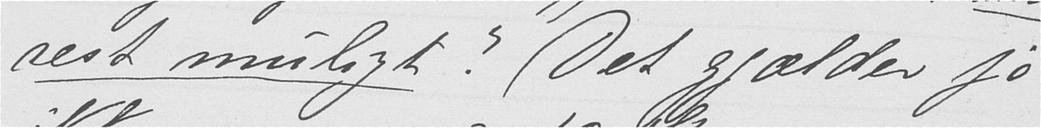rest muligt? Det gjælder jo
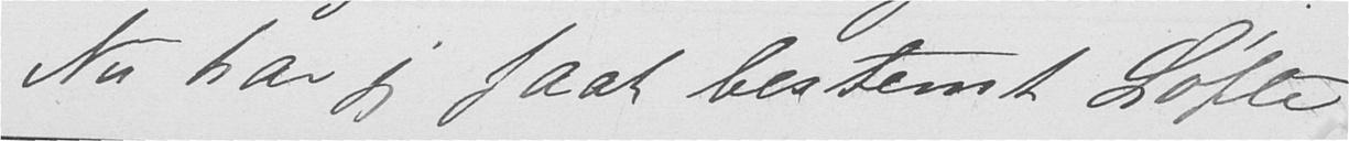Nu har jeg faat bestemt Løfte
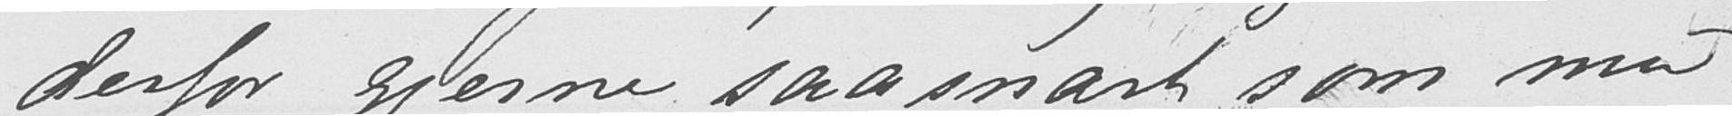derfor gjerne saa snart som mu-
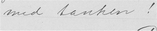med tanken!
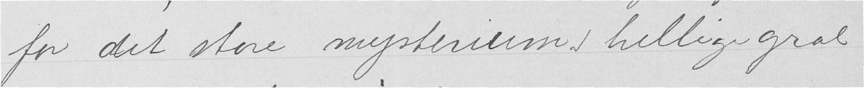for det store mysteriums hellige gral
